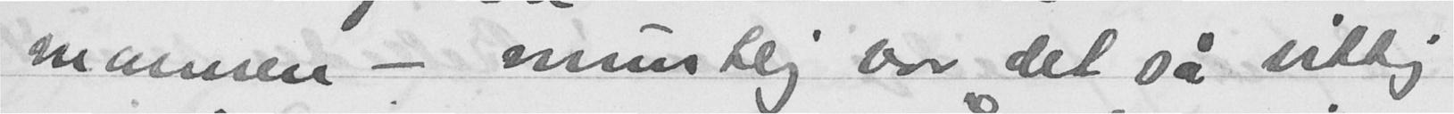mannen - muntlig var det så vittig
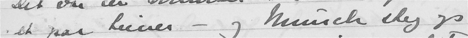et par timer - og Munch steg op
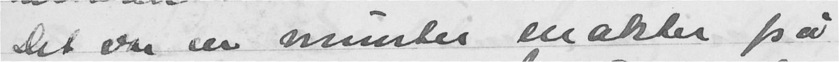Det var en munter enakter på
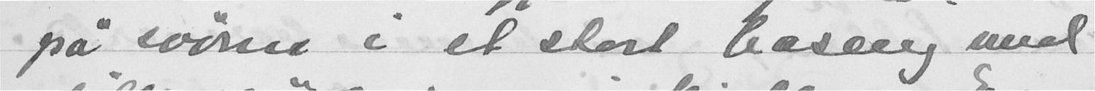på svøm i et stort baseng med
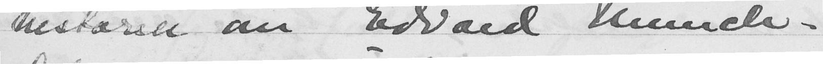historier om Edvard Munch.
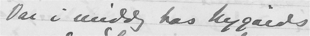Var i middag hos Nygårds
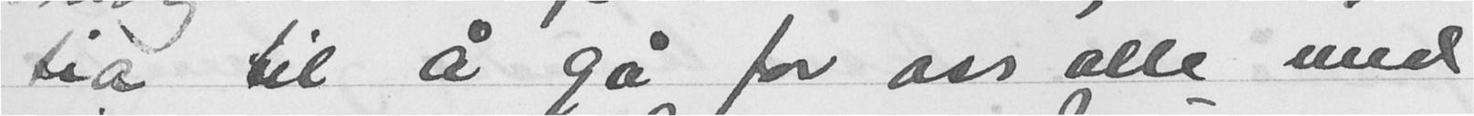tia til å gå for oss alle med
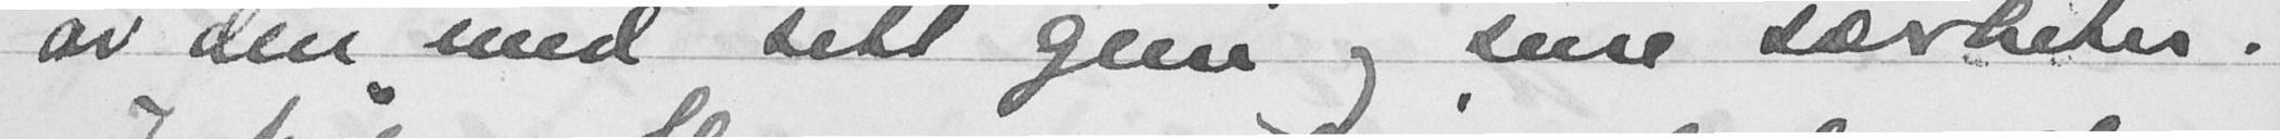av den med sitt geni og sine særheter.
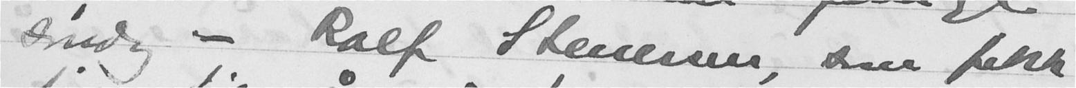søndag - Rolf Stenersen, som fikk
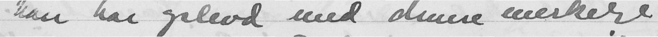han har oplevd med denne merkelige
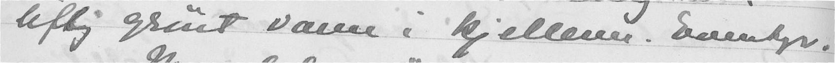liftig grunt vann i kjelleren. Eventyr.
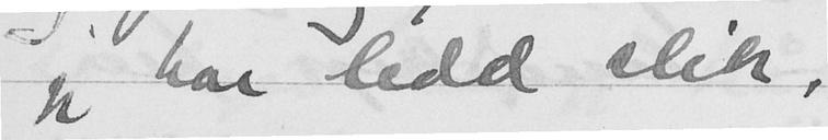jeg har ledd slik.
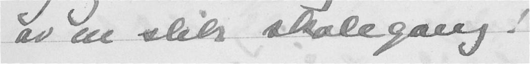av en slik skolegang!
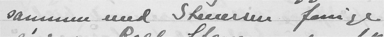sammen med Stenersen forrige
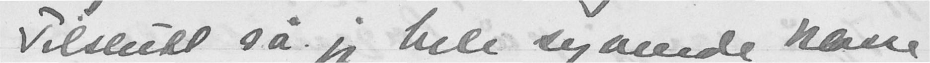Tilslutt så jeg hele syvende klasse
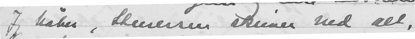Jeg håber, Stenersen skriver ned det,
